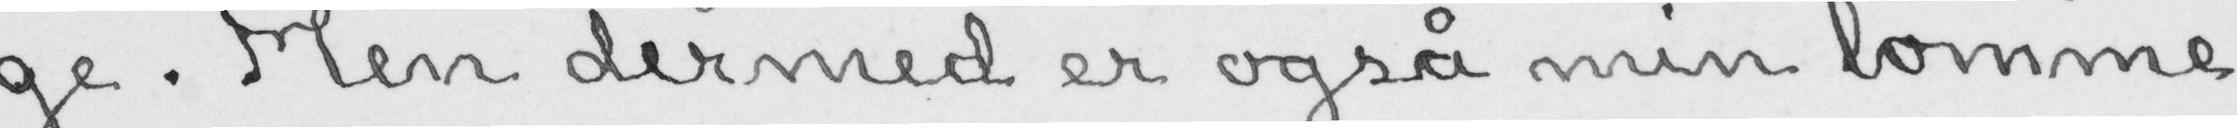ge. Men dermed er også min lomme
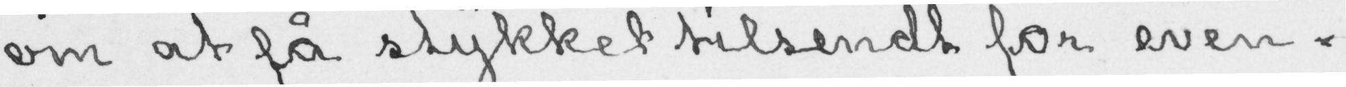om at få stykket tilsendt for even-
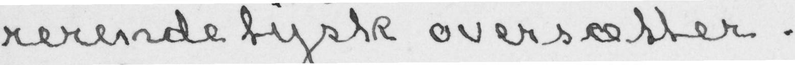rerende tysk oversætter.
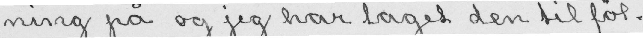ning på og jeg har taget den til føl-
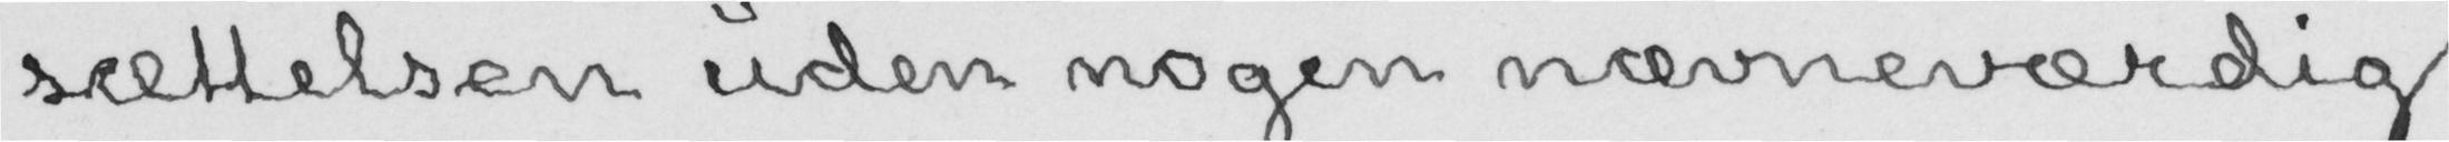sættelsen uden nogen nævneværdig
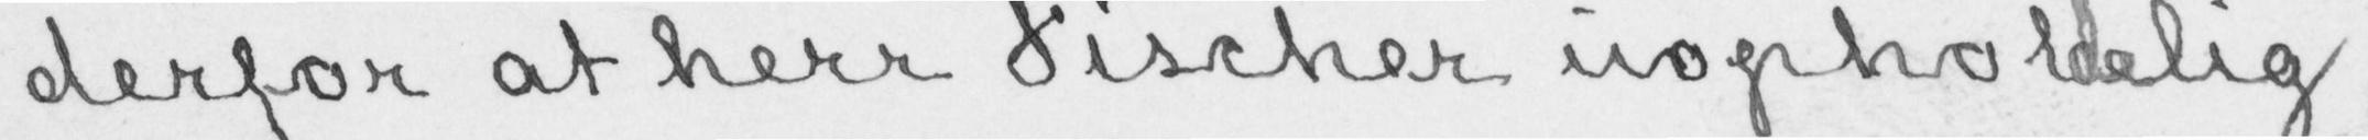derfor at herr Fischer uopholdelig,
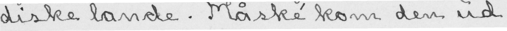diske lande. Måské kom den ud
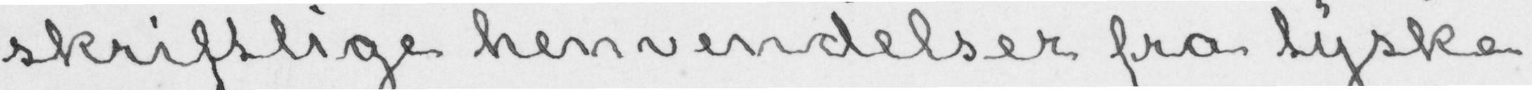skriftlige henvendelser fra tyske
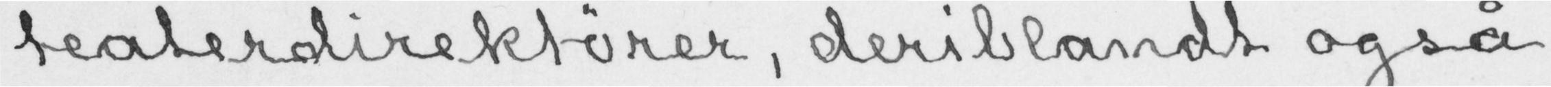teaterdirektører, deriblandt også
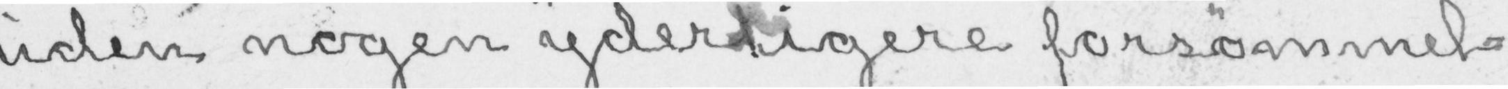uden nogen yderligere forsømmel-
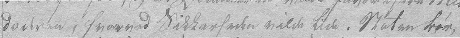dæderen, hvorved Sikkerheden vilde lide. Staten bør
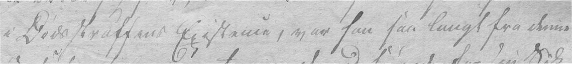i Dødsstraffens Existence, var han saa langt fra denne
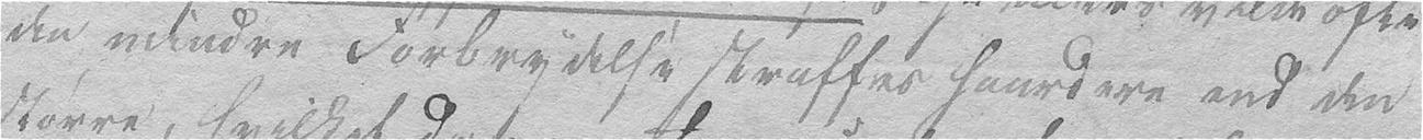den mindre Forbrydelse straffes haardere end den
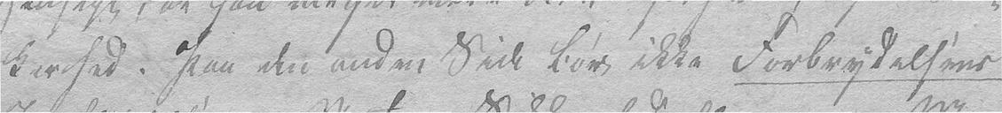kerhed. Paa den anden side bør ikke Forbrydelsens
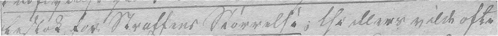lestok for Straffens Størrelse, thi ellers vilde ofte
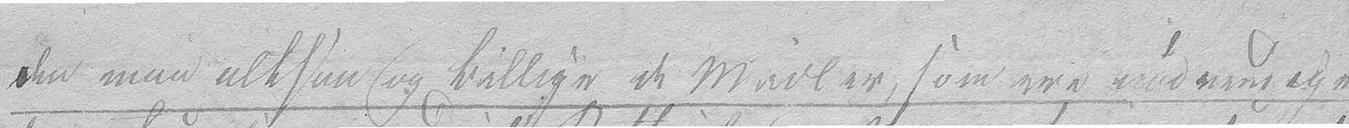den maa altsaa og billige de Midler, som ere nødvendige
